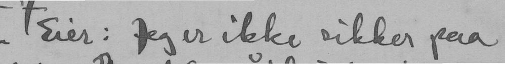Eier: Jeg er ikke sikker paa
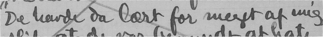De havde da lært for meget af mig
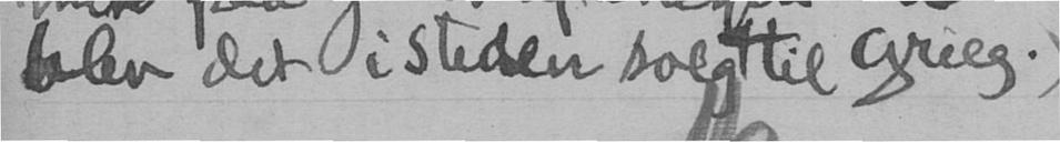blev det i steden solgt til Grieg.)
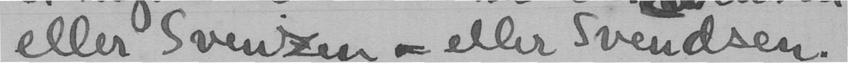eller Svenzen - eller Svendsen.
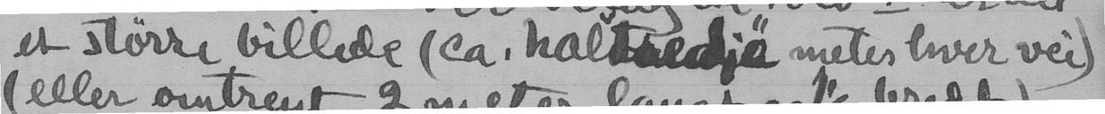et større billede (ca i haldtredje" meter hver vei)
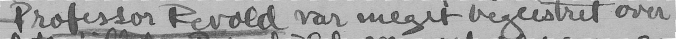Professor Revold var meget begeistret over
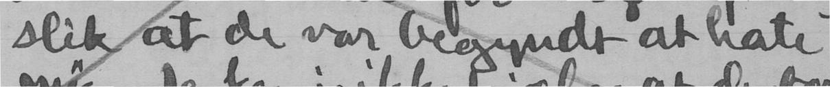slik at de var begyndt at hate
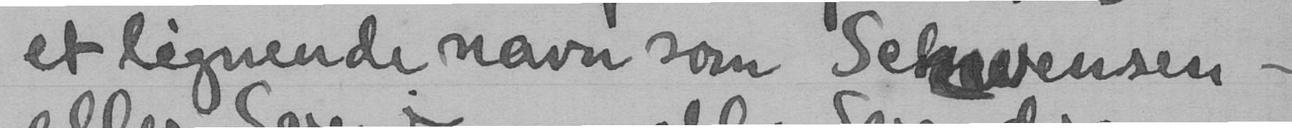et lignende navn som Sehwensen -
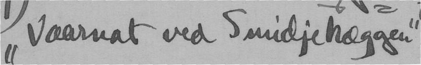"Vaarnat ved Smidjedæggen"
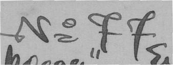N0 77
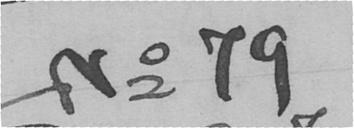No 79
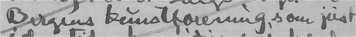Bergens kunstforening, som just
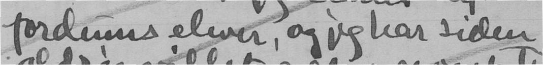fordums elever, og jeg har siden
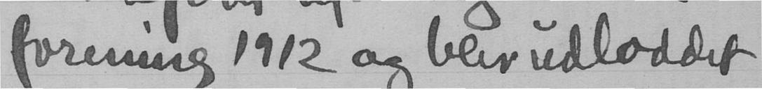forening 1912 og blev udloddet
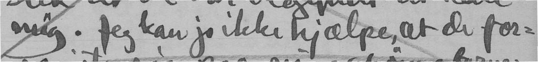mig. Jeg kan jo ikke hjælpe, at de for-
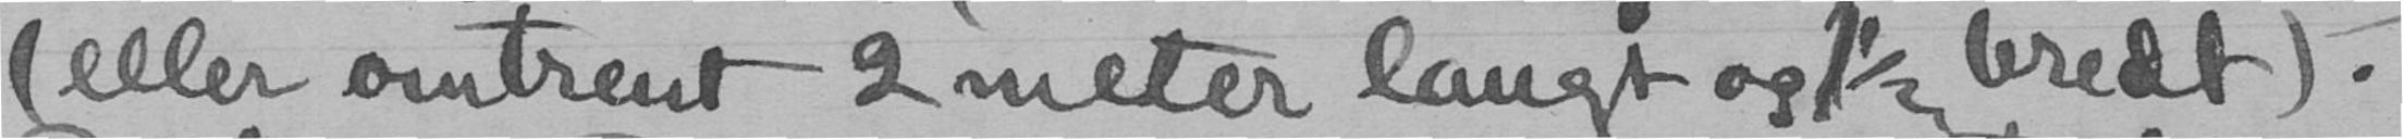(eller omtrent 2meter langt og 1/2 bredt).
